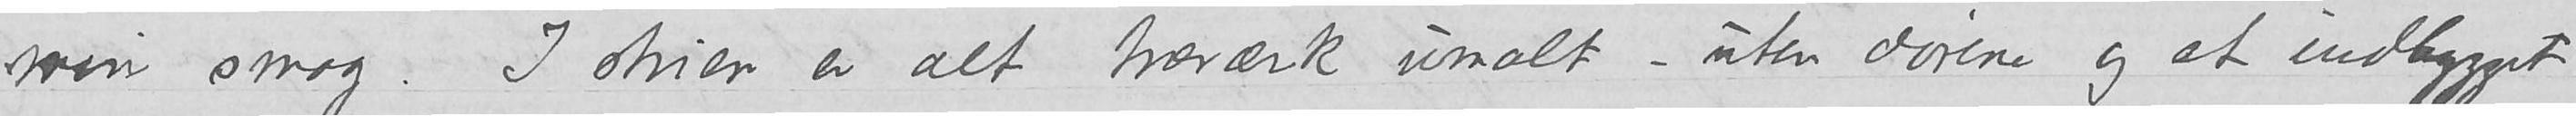min smag. I stuen er alt træværk umalt – uten dørene og et indbygget
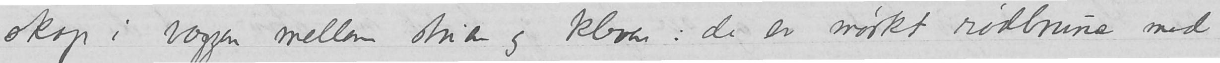skap i væggen mellem stuen og kleven: de er mørkt rødbrune med
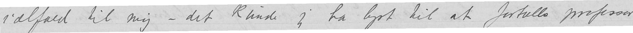ialfald til mig – det kunde jeg ha lyst til at fortælle professor
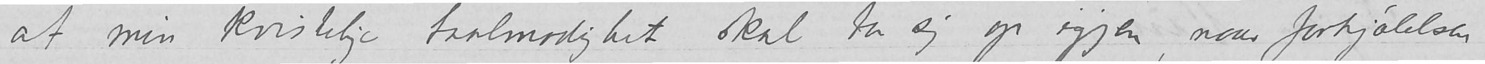at min kristelige taalmodighet skal ta sig op igjen, naar forkjølelsen
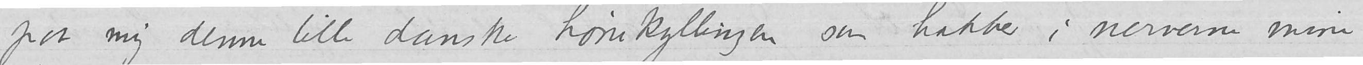paa mig denne lille danske hønekyllingen som hakker i nerverne mine
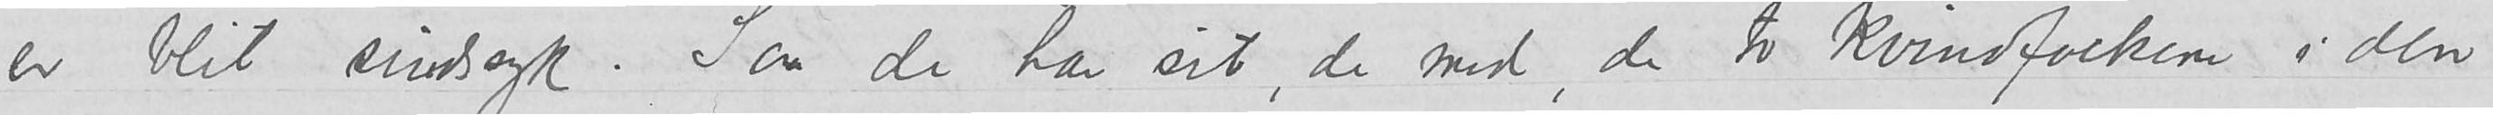er blit sindsyk. Saa de har sit, de med, de to kvindfolkene i den
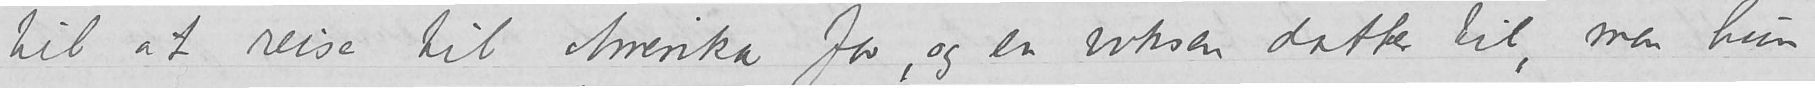til at reise til Amerika for, og en voksen datter til, men hun
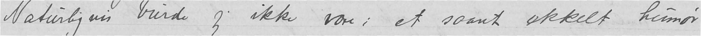Naturligvis burde jeg ikke være i et saant ekkelt humør
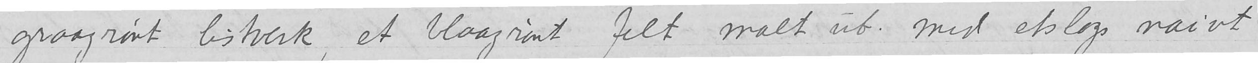graagrønt listverk, et blaagrønt felt malt ut med etslags naivt
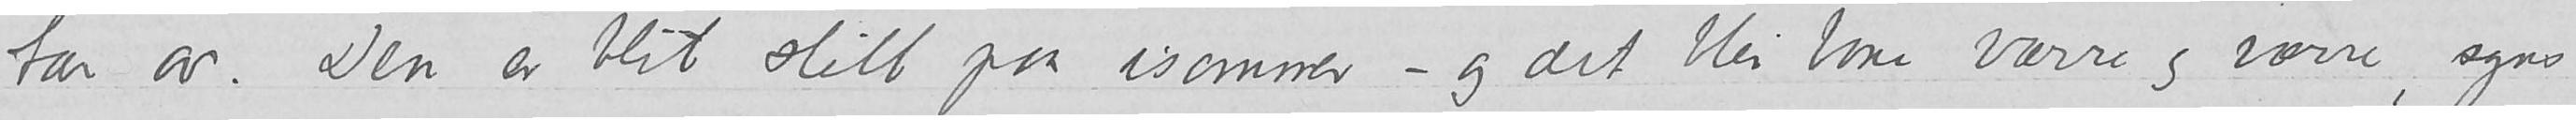tar av. Den er blit slitt paa isommer – og det blir bare værre og værre, syns
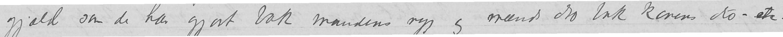gjæld som de har gjort bak mandens ryg og mænds dito bak konens dito – etc.
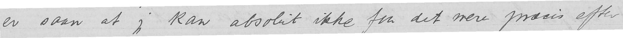er saan at jeg kan absolut ikke faa det mere præcis efter
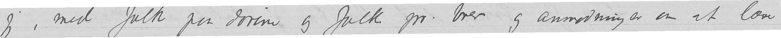jeg, med folk paa dørene og folk pr. brev og anmodninger om at læse
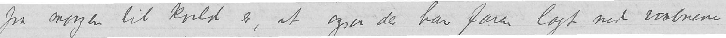fra morgen til kveld er, at ogsaa der har faren lagt ned vaabnene
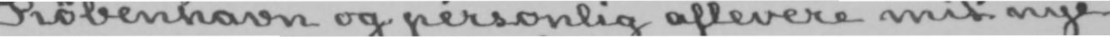København og personlig aflevere mit nye
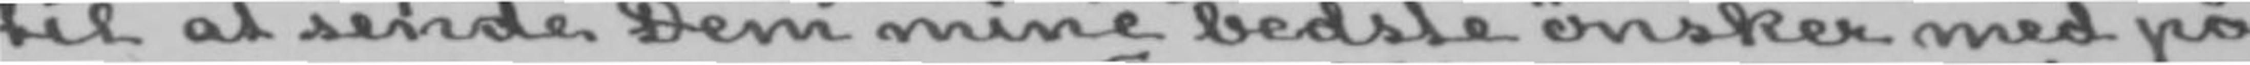til at sende Dem mine bedste ønsker med på
